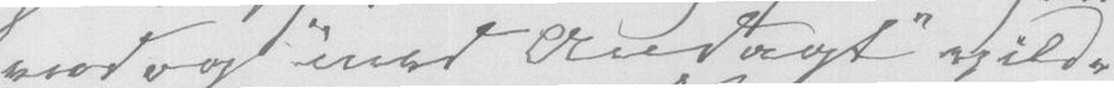endog "med Andagt" vilde
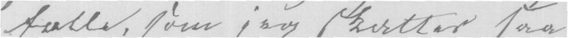fælle, som jeg skatter saa
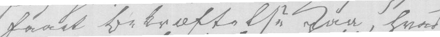faaet bedræftelse paa, hvad
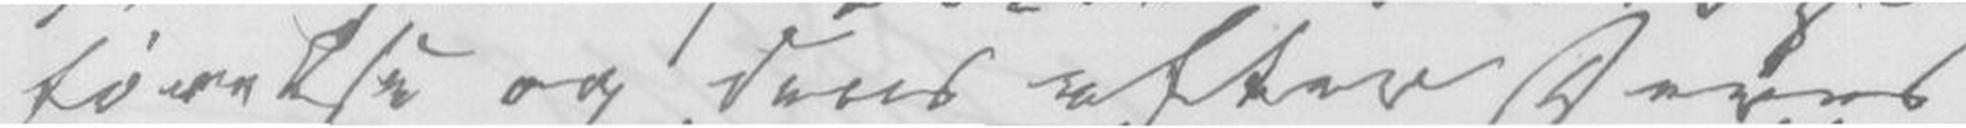førelse og dens efter Deres
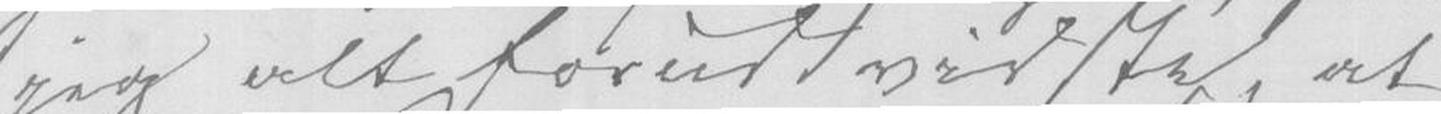jeg alt forud vidste, at
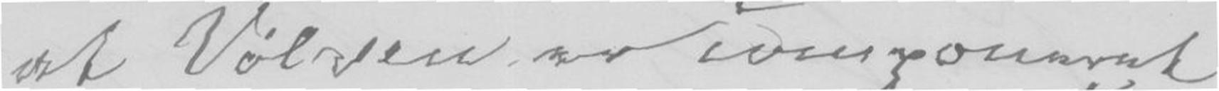at Vølven er Componeret
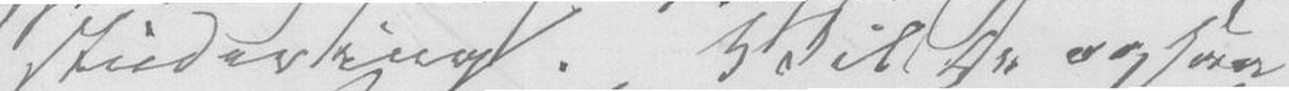studering. Vil De ogsaa
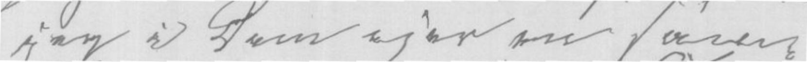jeg i Dem ejer en sam-
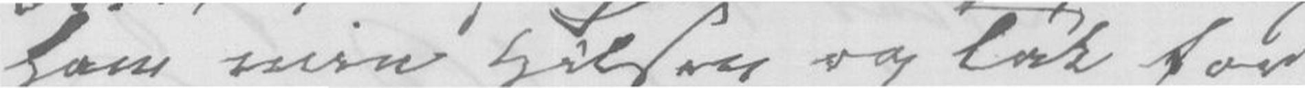ham min Hilsen og Tak for
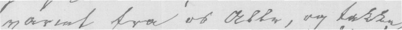varmt fra os Alle, og takke
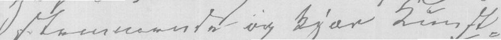stemmende og kjær Kunst-
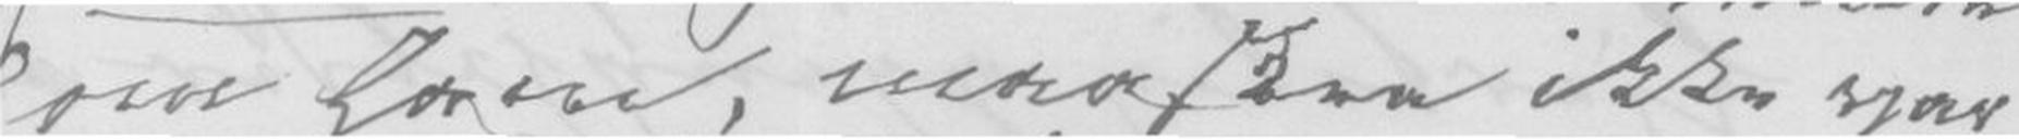om ham, maaskee ikke var
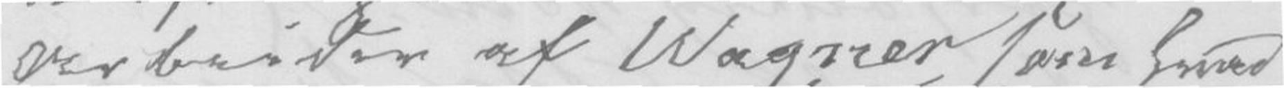Arbeider af Wagner som hvad
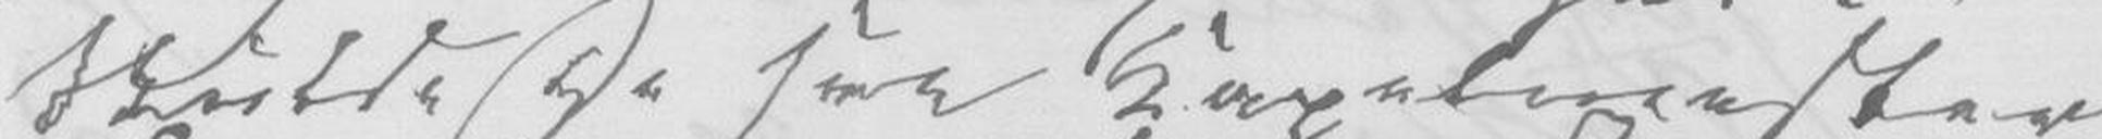Skulde De see Kapelmester
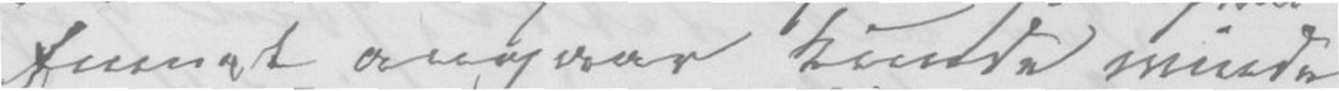Emnet angaar kunde? minde
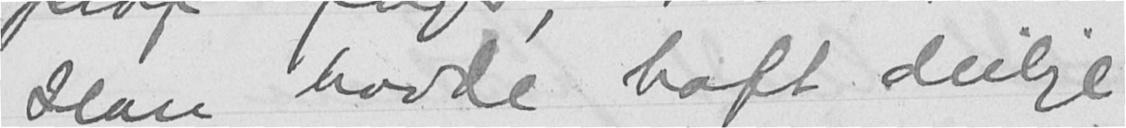Han havde haft deilige
